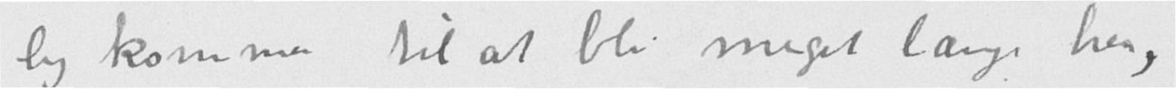lig kommer til at bli meget længe her,
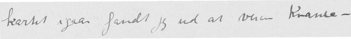kartet i gaar fandt jeg ud at veien Krania-
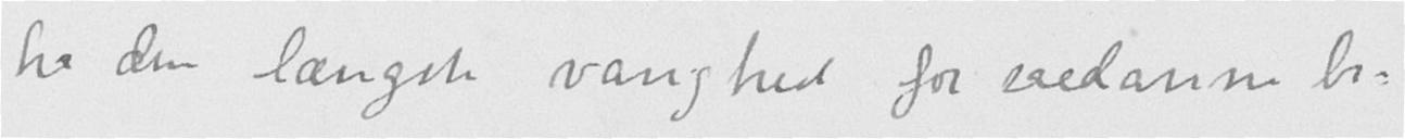ha den længste varighed for saadanne bi-
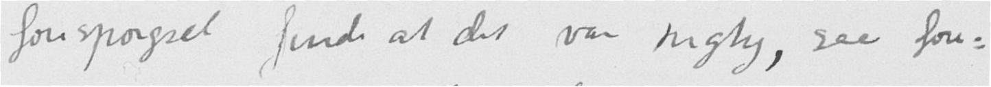forespørgsel finde at det var rigtig, saa fore-
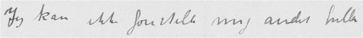Jeg kan ikke forestille mig andet heller
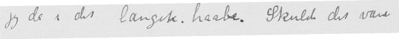jeg da i det længste haabe. Skulde det være
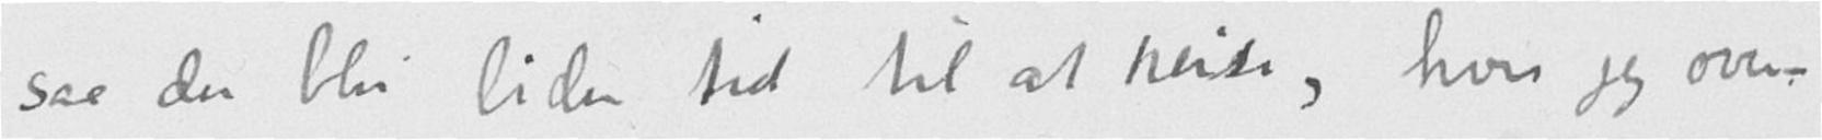saa der blir liden tid til at reise, hvis jeg over-
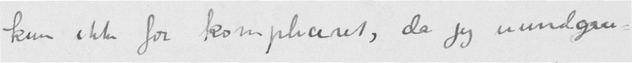kun ikke for kompliceret, da jeg uundgaae-
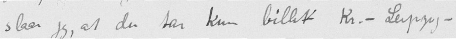slaar jeg, at du tar kun billett Kr.-Leipzig-
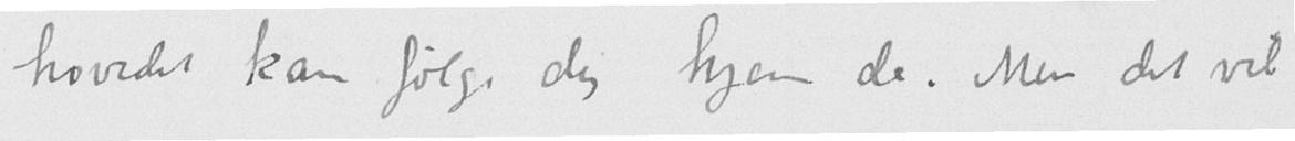hovedet kan følge dig hjem da. Men det vil
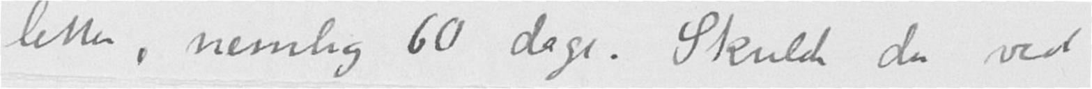letter, nemlig 60 dage. Skulde du ved
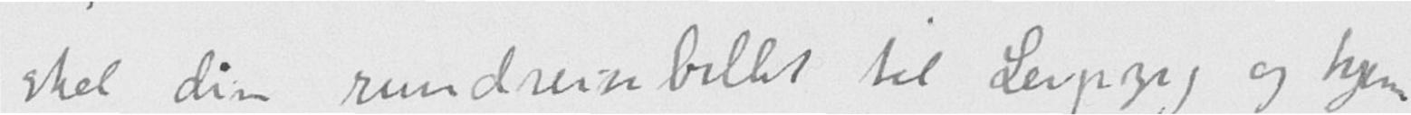skal din rundreisebillet til Leipzig og hjem
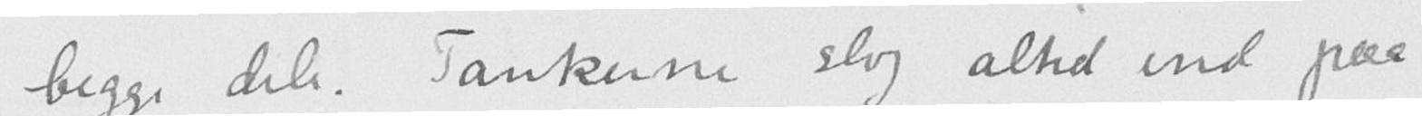begge dele. Tankerne slog altid ind paa
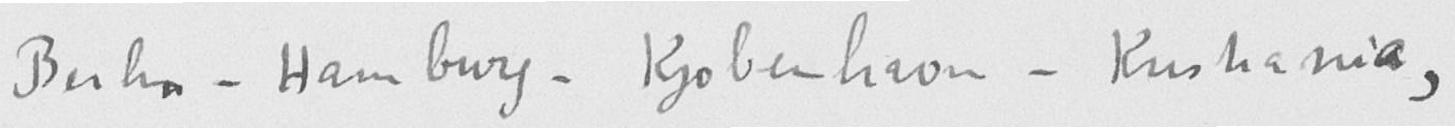Berlin-Hamburg-Kjøbenhavn-Kristiania,
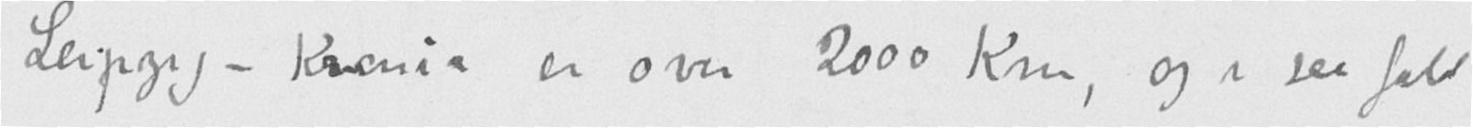Leipzig-Krania er over 2000 Km, og i saa fald
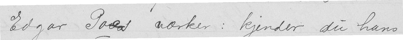Edgar Poes værker: kjender du hans
